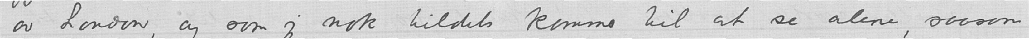av London, og som jeg nok tildels kommer til at se alene, saasom
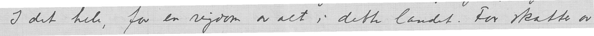I det hele, for en rigdom av alt i dette landet. For skatter av
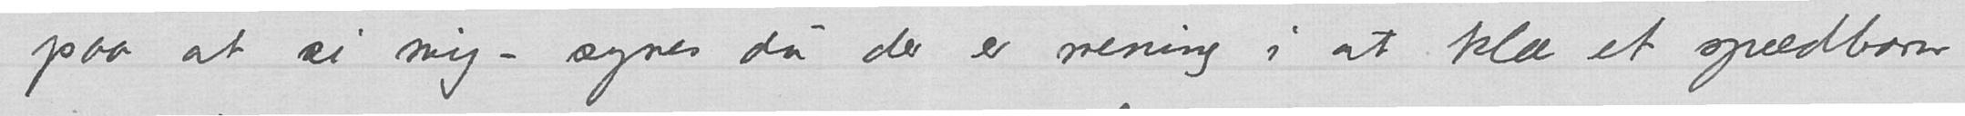paa at si mig - synes du der er mening i at klæ et spædbarn
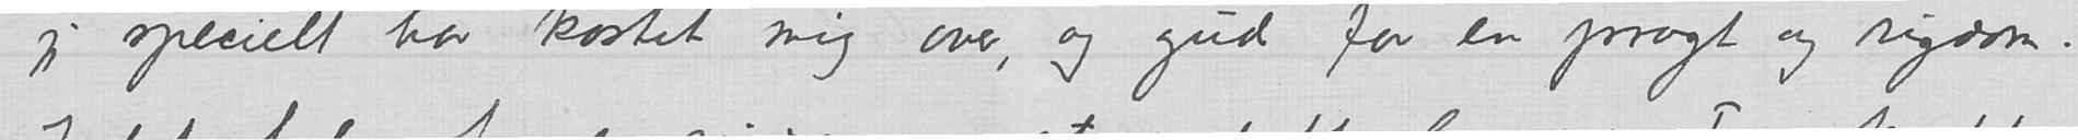jeg specielt har kastet mig over, og Gud for en pragt og rigdom.
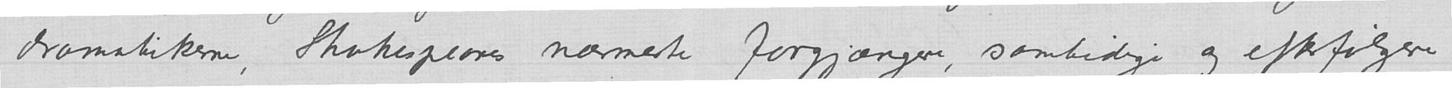dramatikerne, Shakespeares nærmeste forgjængere, samtidige og efterfølgere
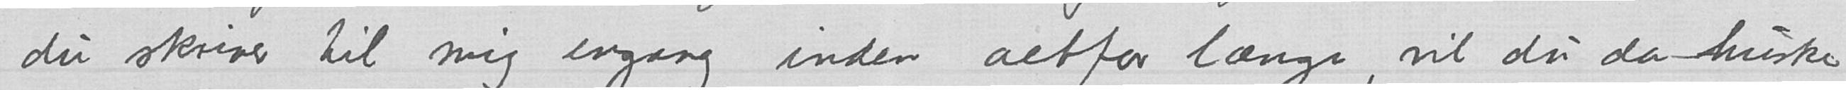du skriver til mig engang inden altfor længe, vil du da huske
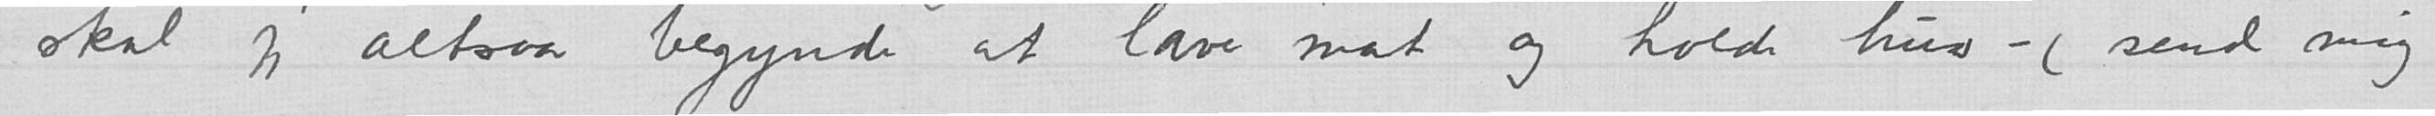skal jeg altsaa begynde at lave mat og holde hus - (send mig
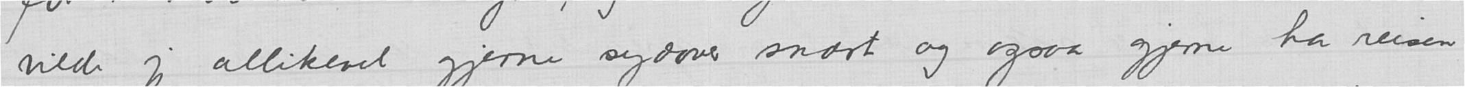vilde jeg allikevel gjerne sydover snart og ogsaa gjerne ha reisen
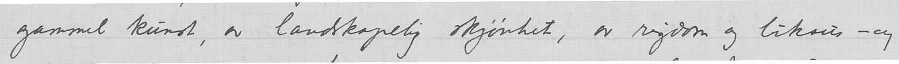gammel kunst, av landskapelig skjønhet, av rigdom og luksus - og
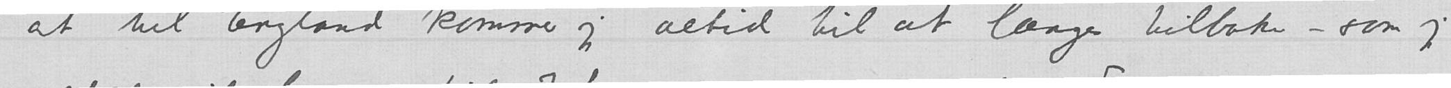at til England kommer jeg altid til at længes tilbake - som jeg
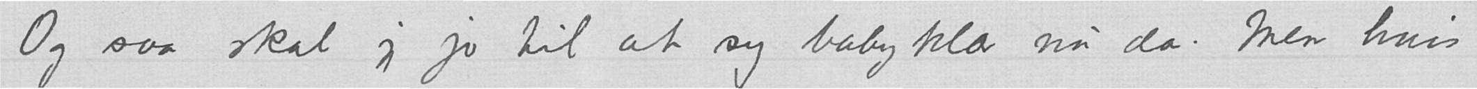Og saa skal jeg jo til at sy babyklær nu da. Men hvis
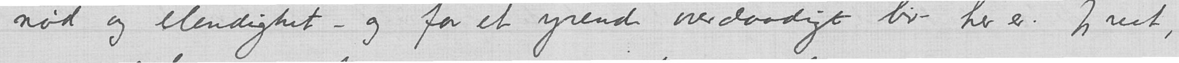nød og elendighet - og for et yrende overdaadigt liv her er. Jeg vet,
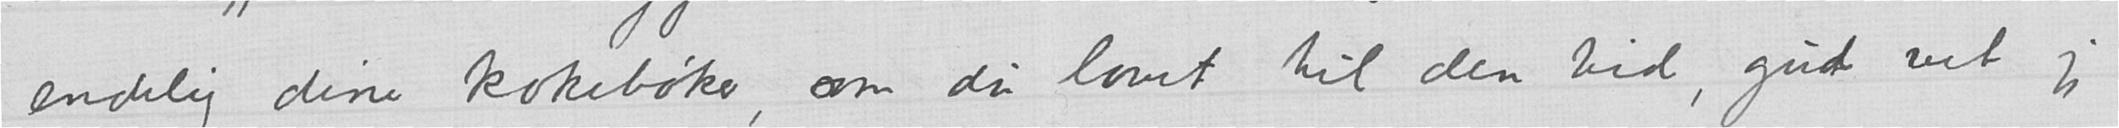endelig dine kokebøker, som du lovet til den tid, gud vet jeg
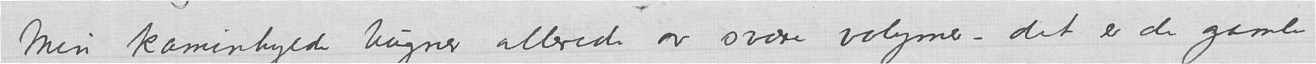Min kaminhylde bugner allerede av svære volymer - det er de gamle
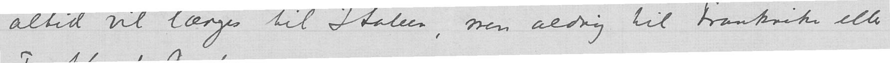altid vil længes til Italien, men aldrig til Frankrike eller
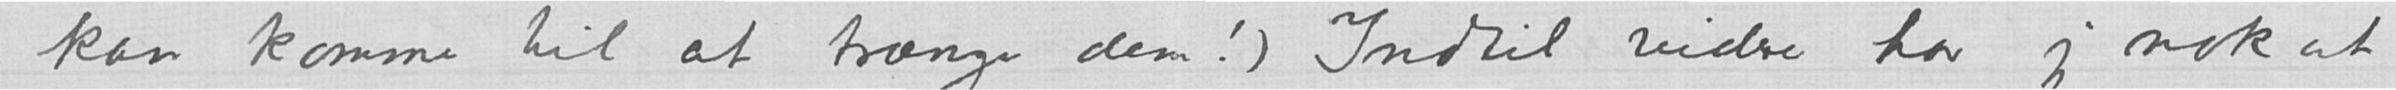kan komme til at trænge dem!) Indtil videre har jeg nok at
